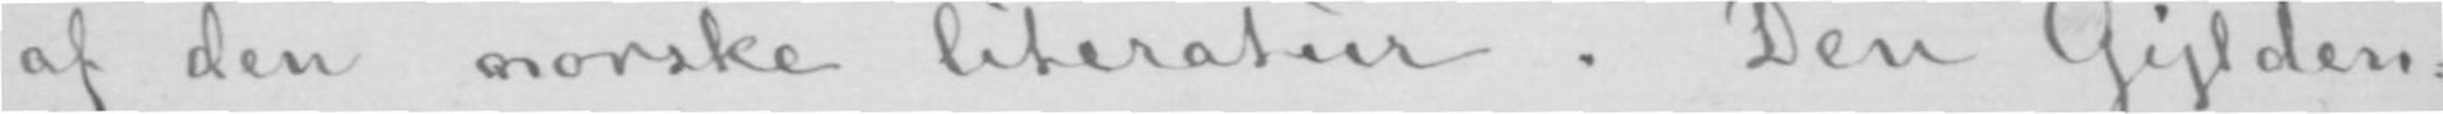af den norske literatur. Den Gylden-
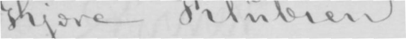Kjære Klubien!
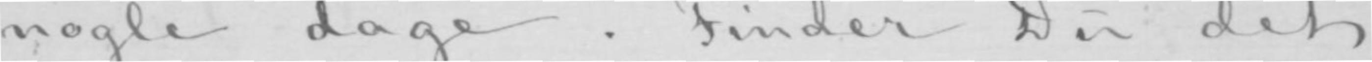nogle dage. Finder Du det
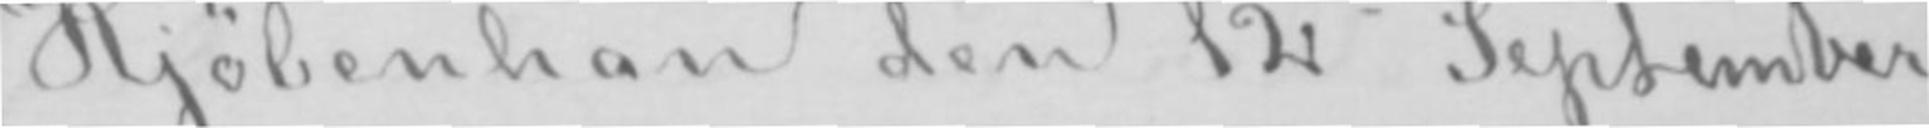Kjøbenhan den 12te September
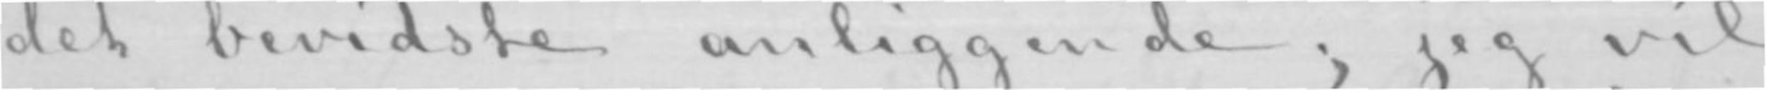det bevidste anliggende; jeg vil
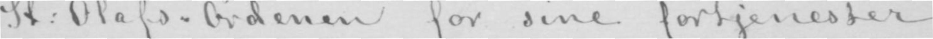St: Olafs-Ordenen for sine fortjenester
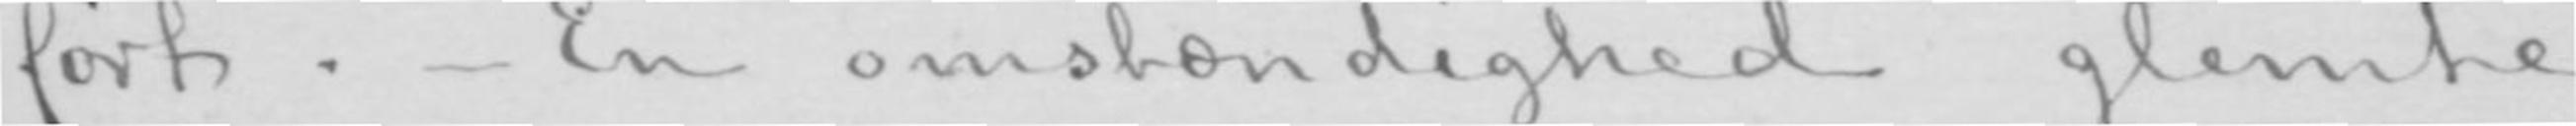ført.- En omstændighed glemte
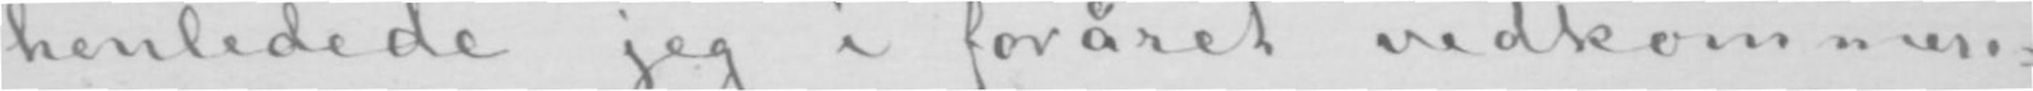henledede jeg i foråret vedkommen-
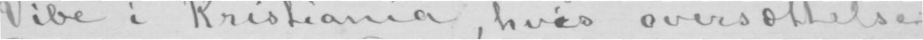Vibe i Kristiania, hvis oversættelse
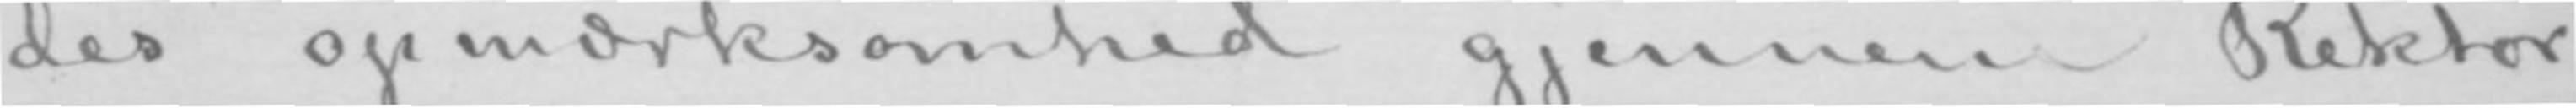des opmærksomhed gjennem Rektor
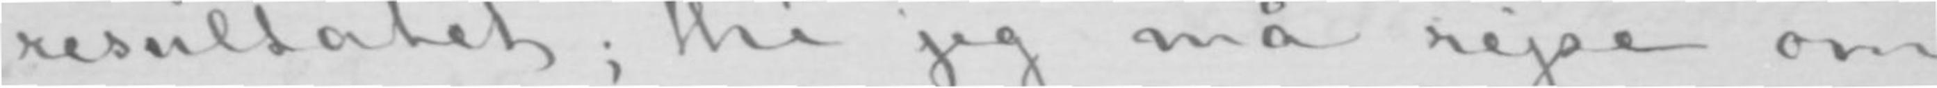resultatet; thi jeg må rejse om
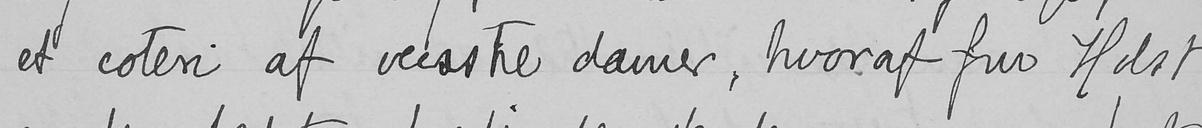et coteri af veesstre damer, hvoraf fru Holst
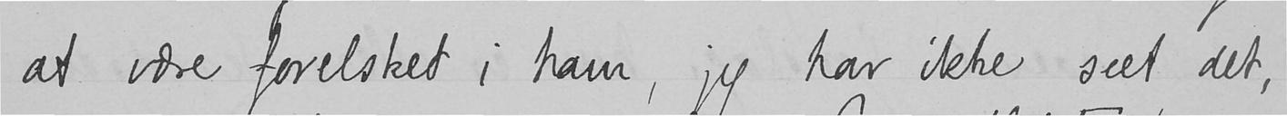at være forelsket i ham, jeg har ikke seet det,
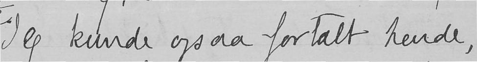Jeg kunde ogsaa fortalt hende,
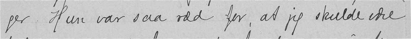ger. Hun var saa ræd for, at jeg skulde være
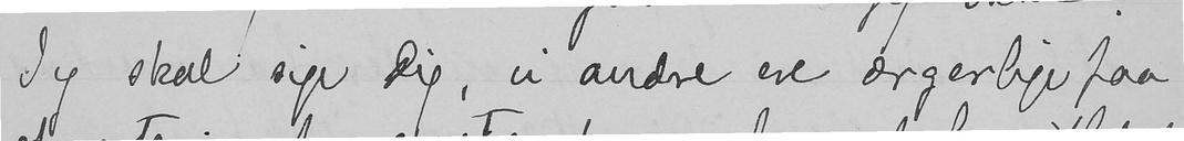Jeg skal sige Dig, vi andre ere ærgerlige paa
